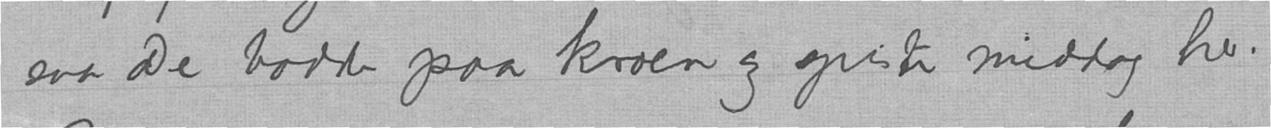saa De bodde paa kroen og spiste middag her.
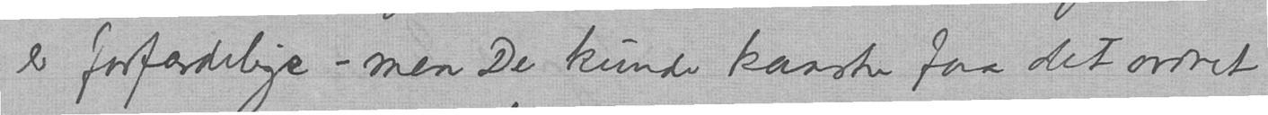er forfærdelige – men De kunde kanske faa det ordnet
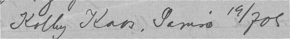Kolby Kaas, Samsø 19/7 09
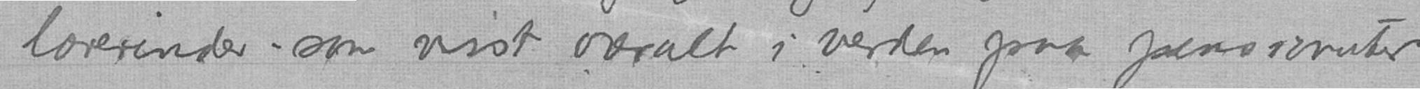lærerinder - som vist overalt i verden paa pensionater
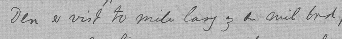Den er vist to mile lang og en mil bred,
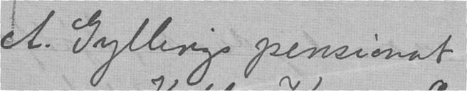A. Gyllings pensionat
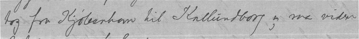tog fra Kjøbenhavn til Kallundborg og saa videre
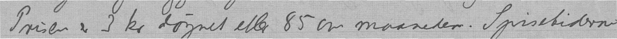Prisen er 3 kr døgnet eller 85 om maanedem. Spisetiderne
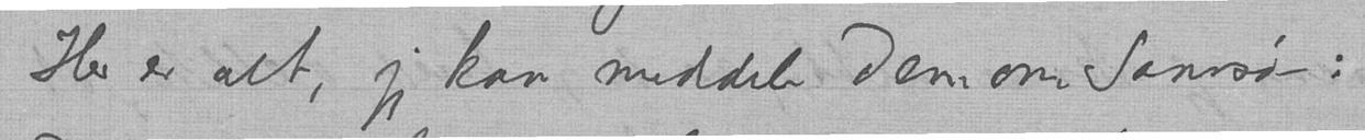Her er alt, jeg kan meddele Dem om Samsø - :
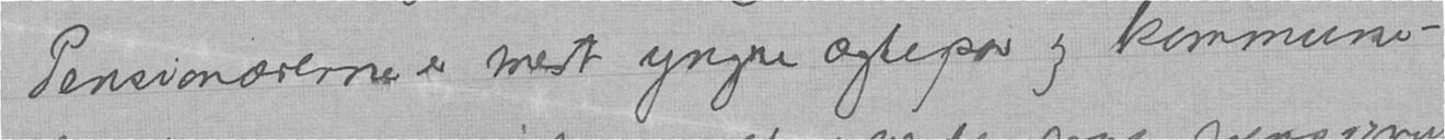Pensionærerne er mest yngre ægtepar og kommune-
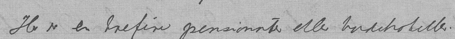Her er en trefire pensionater eller badehoteller.
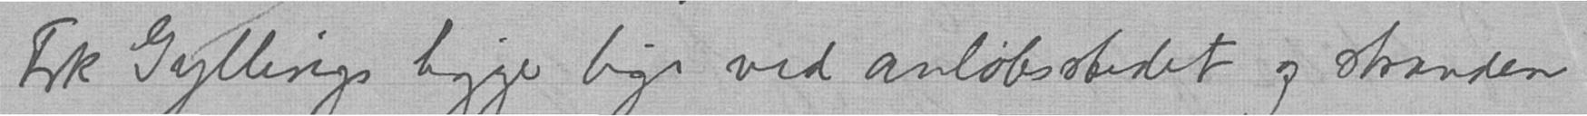Frk Gyllings ligger lige ved anløbsstedet og stranden
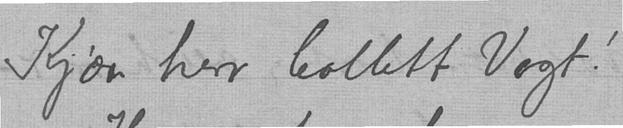Kjære herr Collett Vogt!
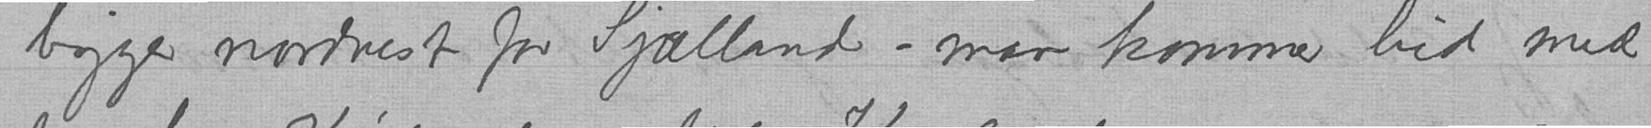ligger nordvest for Sjælland – man kommer hid med
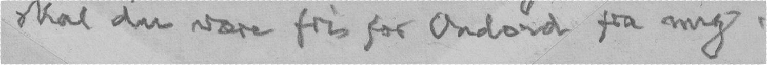skal du være fri for Ondord fra mig.
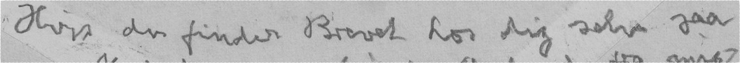Hvis du finder Brevet hos dig selv saa
